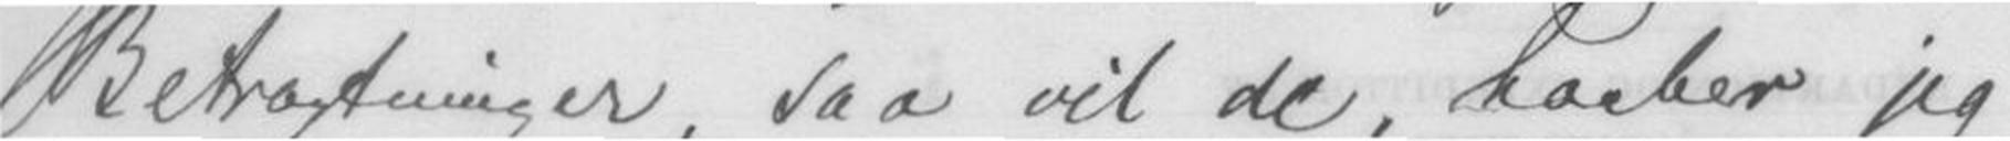Betragtninger, saa vil de, haaber jeg
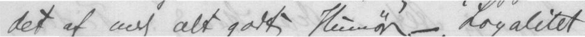det af alt godt Humør-, Lojalitet
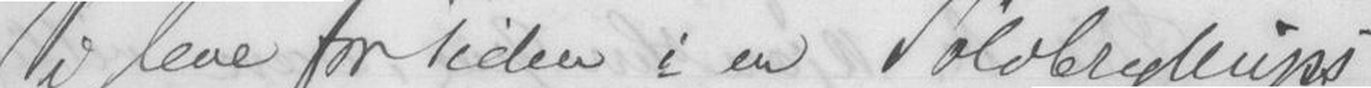Vi lever for Tiden i en Sølvbryllups-
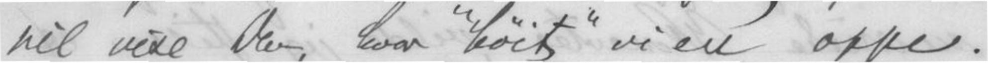vil veie , hvor "høit" vi er oppe.
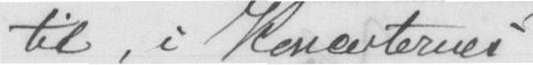til, i Koncerterners
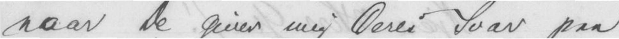naar De giver mig Deres Svar paa
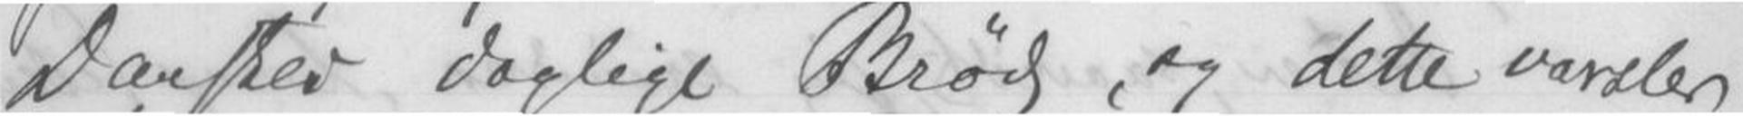daglige Brød, og dette varsler
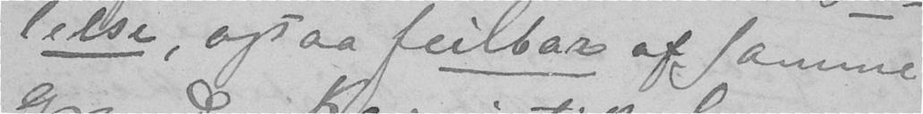lelse, ogsaa feilbar af samme
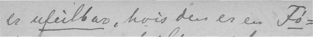er ufeilbar, hvis den er en Fø-
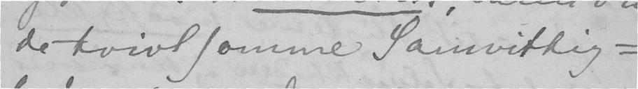de tvivlsomme Samvittig-
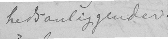hedsanliggender.
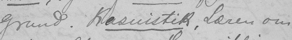Grund. Kasuistik, Læren om
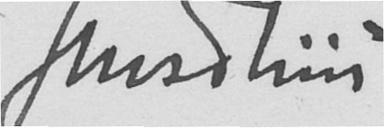Jens Thiis
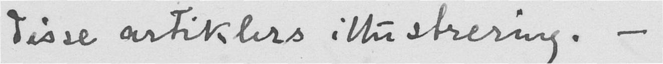disse artiklers illustrering. -
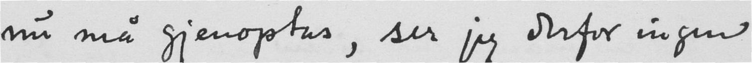nu må gjenoptas, ser jeg derfor ingen
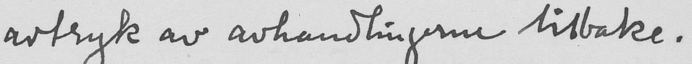avtryk av avhandlingerne tilbake.
